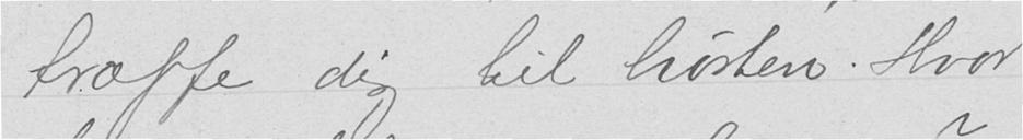træffe dig til høsten. Hvor
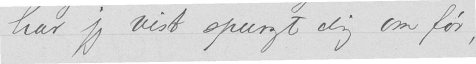har jeg vist spurgt dig om før,
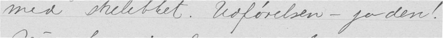med skelettet. Udførelsen – ja den!
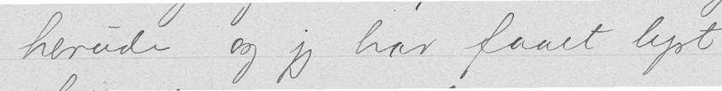herude og jeg har faaet lyst
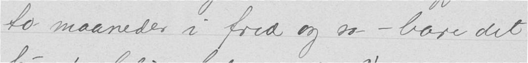to maaneder i fred og ro - bare det
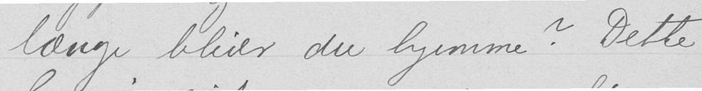længe bliver du hjemme? Dette
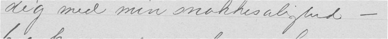dig med min snakkesalighed -
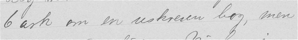6 ark om en uskreven bog, men
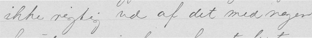ikke rigtig ud af det med nogen
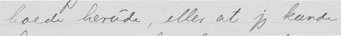boede herude, eller at jeg kunde
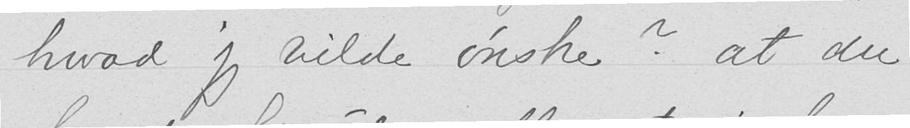hvad jeg vilde ønske? at du
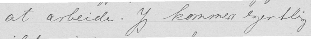at arbeide. Jeg kommer egentlig
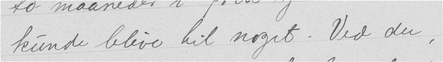kunde blive til noget. Ved du,
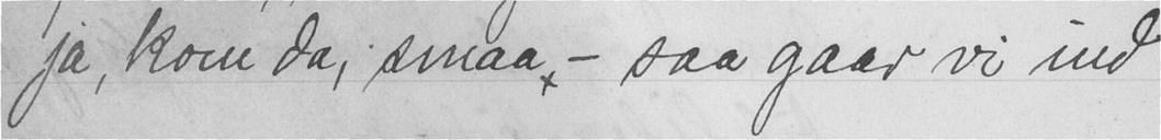ja, kom da, smaa, - saa gaar vi ind
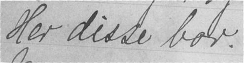Her disse bor.
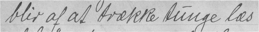blir af at trække tunge læs
